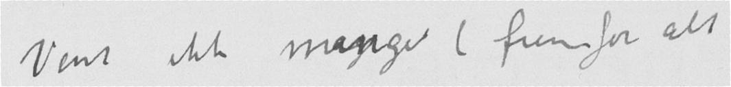Vent ikke mange (fremfor alt
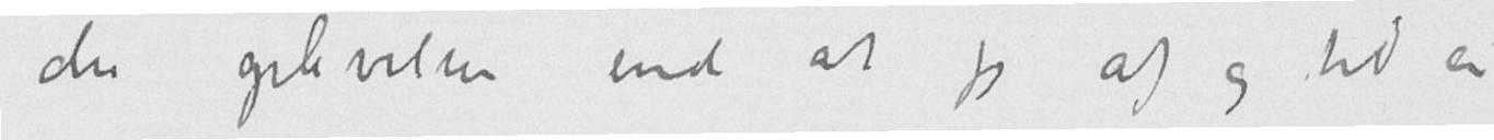dre oplevelser end at jeg af og til er
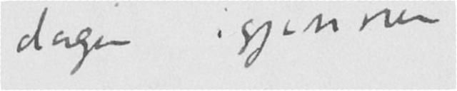dagen igjennem
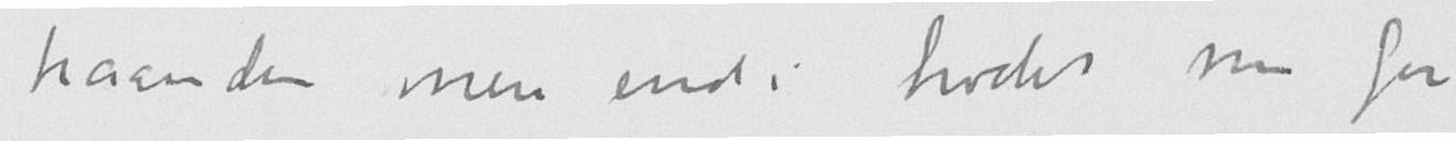haanden mere end i hodet nu for
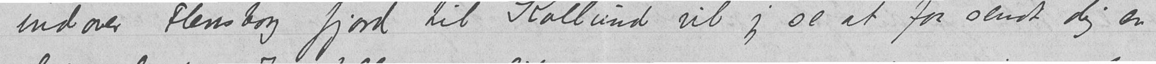indover Flensborg fjord til Kallund vil jeg se at faa sendt dig en
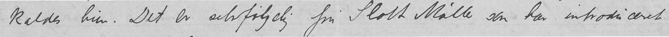kaldes hun. Det er selvfølgelig fru Slott Møller som har introduceret
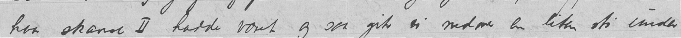hvor skanse ll hadde været og saa gik vi nedover en liten sti under
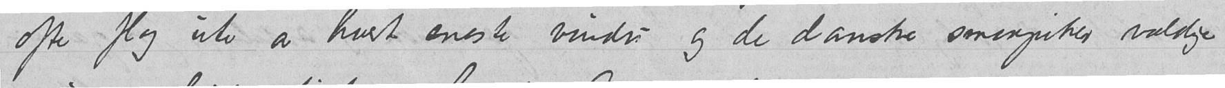ofte flag ute av hvert eneste vindu og de danske smaapiker vældige
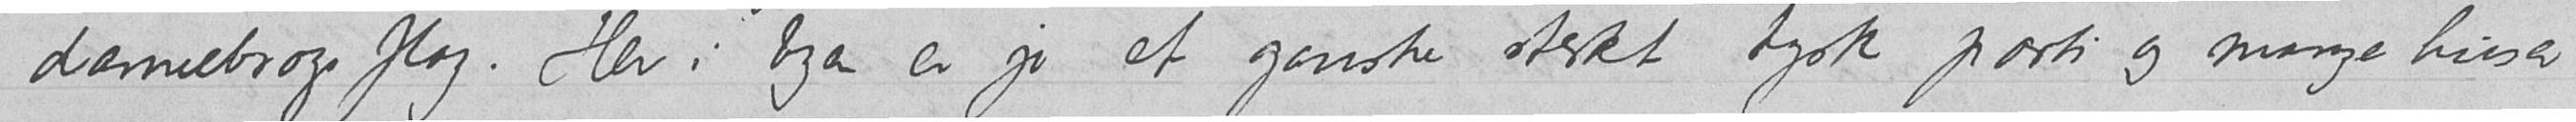dannebrogsflag. Her i byen er jo et ganske sterkt tysk parti og mange huser
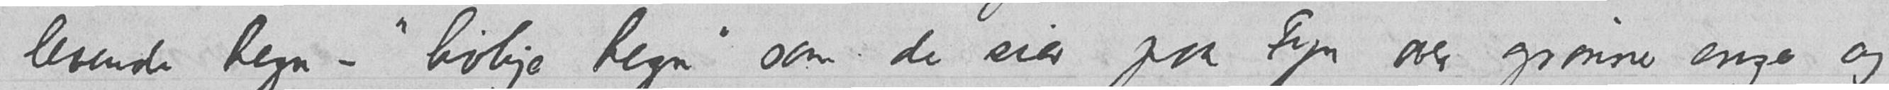levende hegn – "livlige hegn" som de sier paa Fyn over grønne enge og
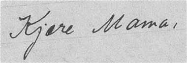Kjære Mama,
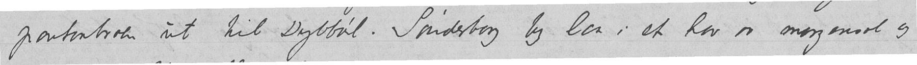pontonbroen ut til Dybbøl. Sønderborg by laa i et hav av morgensol og
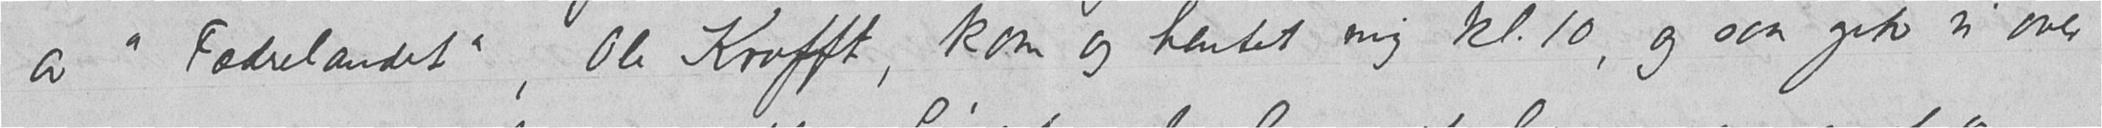av «Fædrelandet», Ole Krafft , kom og hentet mig kl. 10, og saa gik vi over
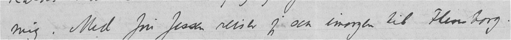mig. Med fru Jessen reiser jeg saa imorgen til Flensborg.
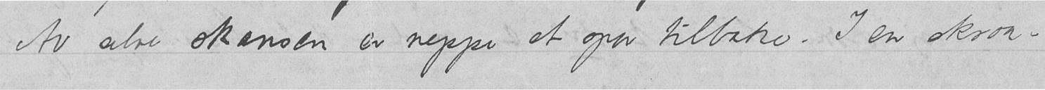Av selve skansen er neppe et spor tilbake. I en skraa-
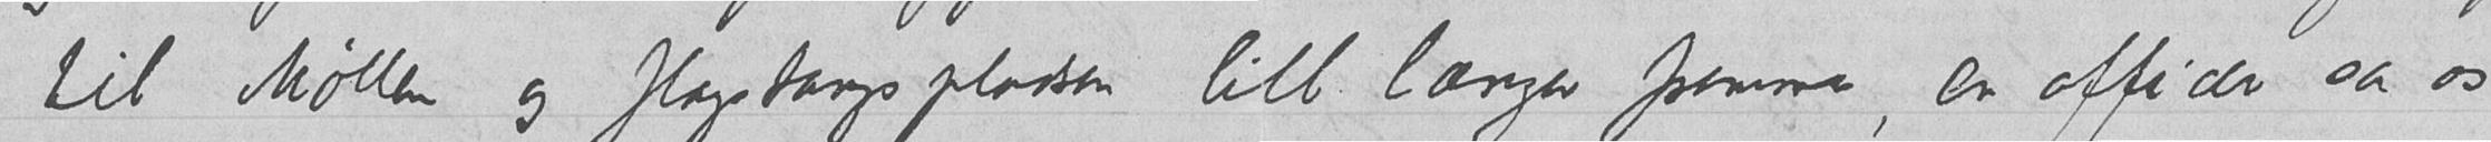til Møllen og flagstangspladsen litt længer fremme, en officer sa os
